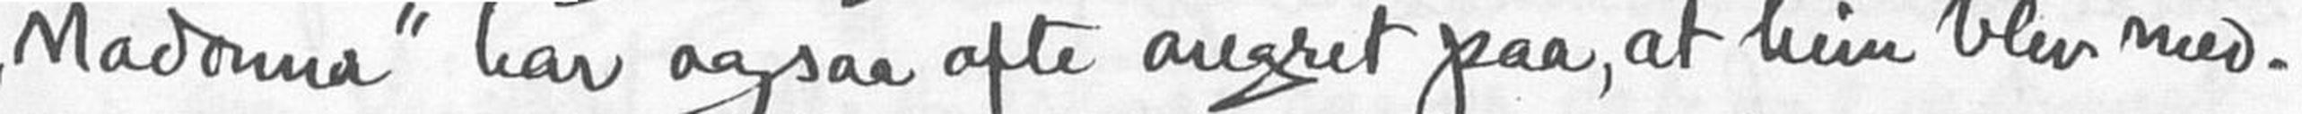"Madonna" har ogsaa ofte angret paa, at hun blev med.
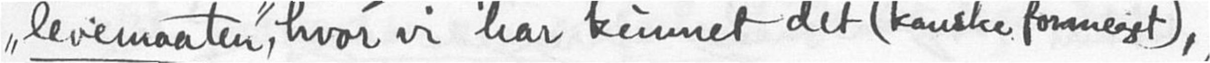"levemaaten", hvor vi har kunnet det (kanske formeget).
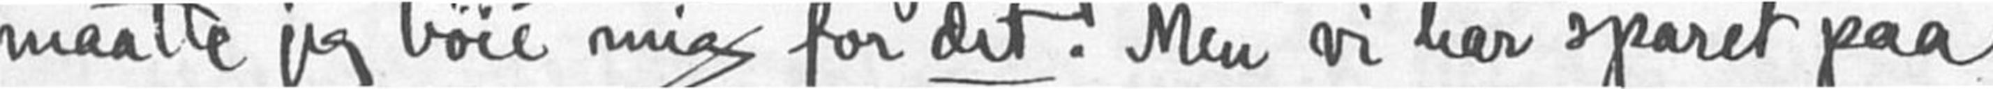maatte jeg bøie mig for det. Men vi har sparet paa
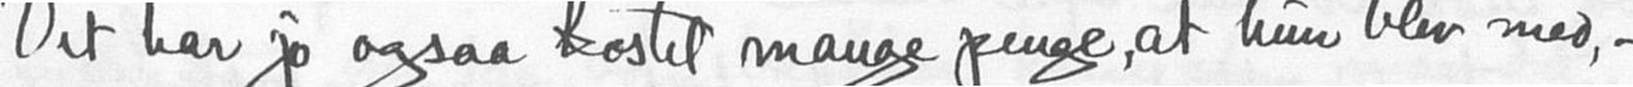Det har jo ogsaa kostet mange penge, at hun blev med, -
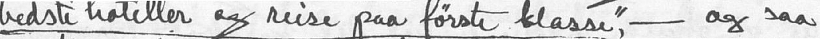bedste hoteller og reise paa første klasse", - og saa
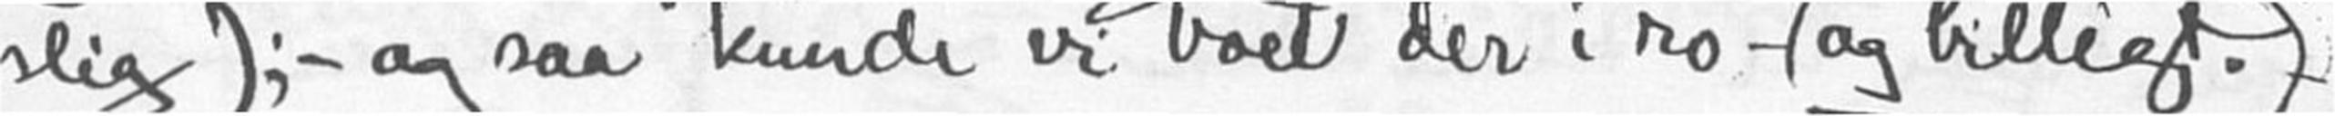slig); - og saa kunde vi boet der i ro - (og billigt).
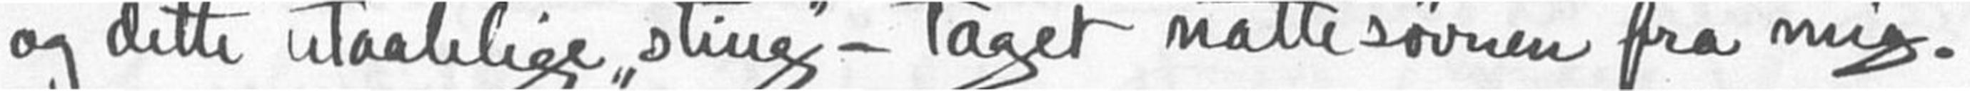og dette utaalelige "sting" - taget nattesøvnen fra mig.
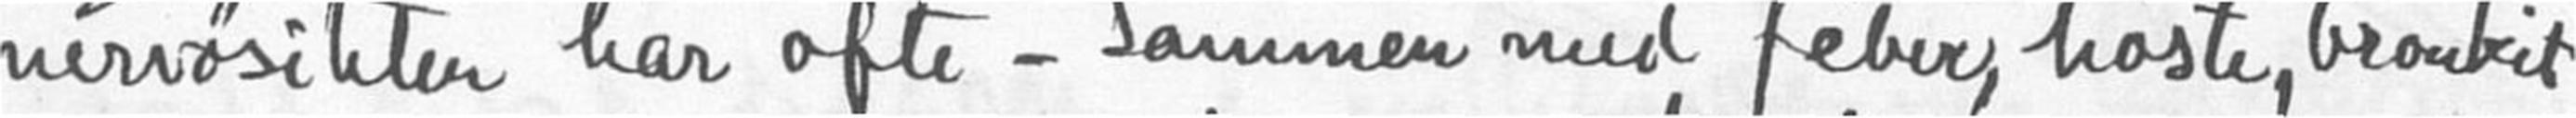nervøsiteten har ofte - sammen med feber, hoste, bronkit
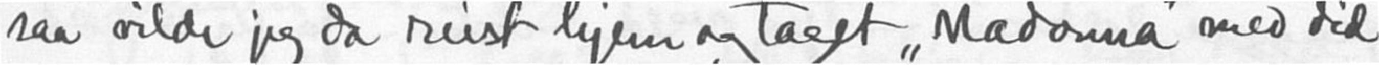saa vilde jeg da reist hjem og taget "Madonna" med did
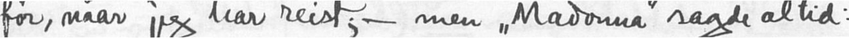for, naar jeg har reist, - men "Madonna" sagde altid
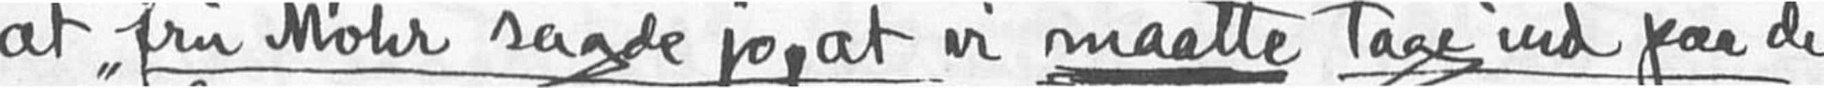at "fru Mohr sagde jo, at vi maatte tage ind paa de
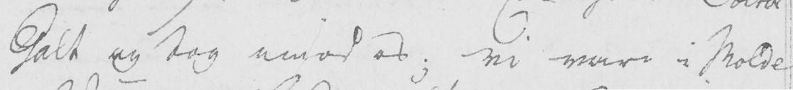Galt og tog imod os; vi vare i Molde
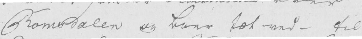Romsdalen og boer tæt ved - til
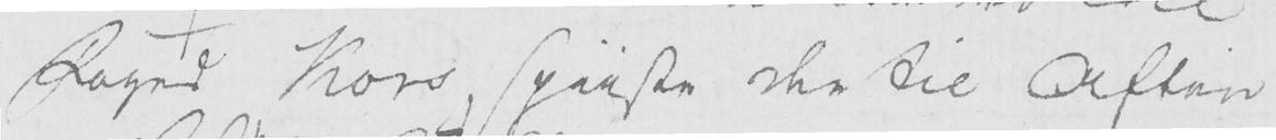Foged Kors, spiiste der til Aften
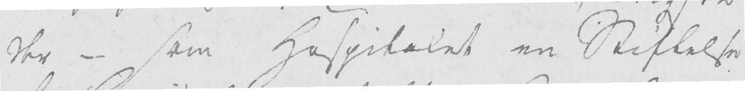der - som Hospitalet en Stiftelse
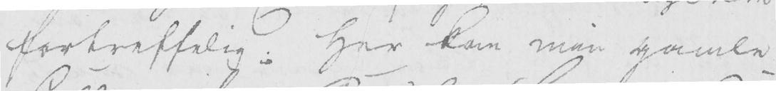fortreffelig. Her kom min gamle
